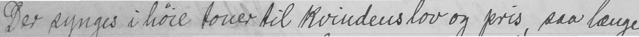Der synges i høie toner til kvindens lov og pris, saa længe
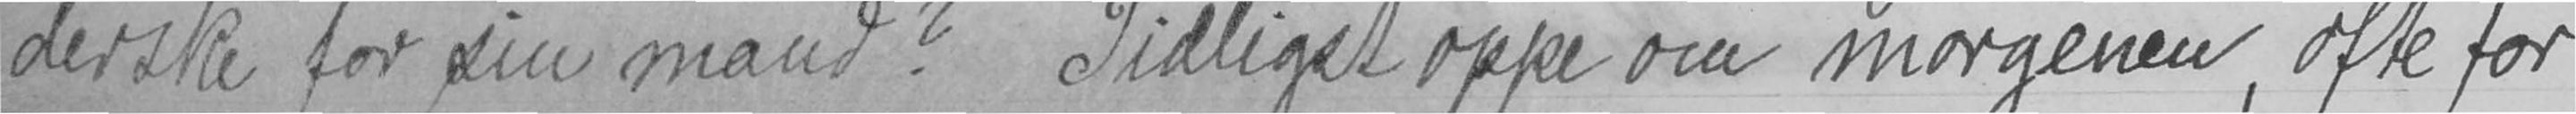derske for sin mand? Tidligst oppe om morgenen, ofte for
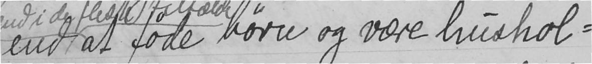end at føde børn og være hushol-
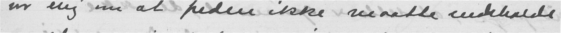var enig om at freden ikke maatte indeholde
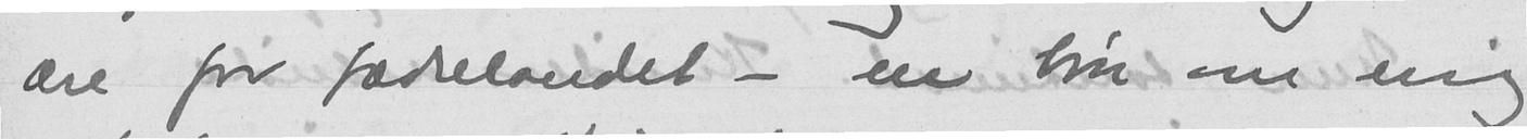ære for fædrelandet - en bru om evig
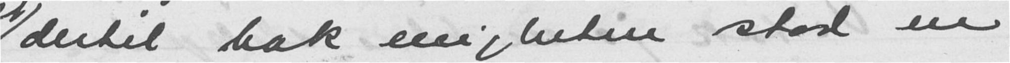dertil bak enigheten stod en
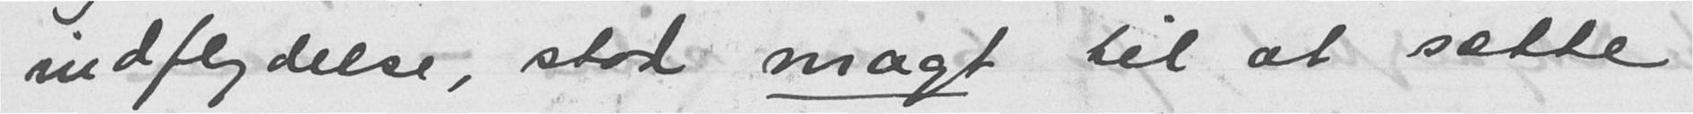indflydelse, stod magt til at sætte
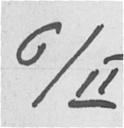6/II
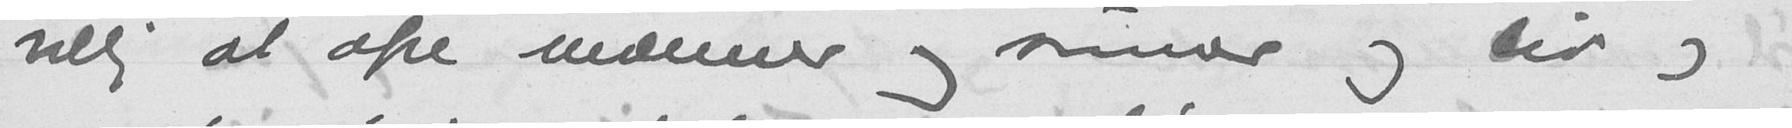villig at ofre mænner og sønner og liv og
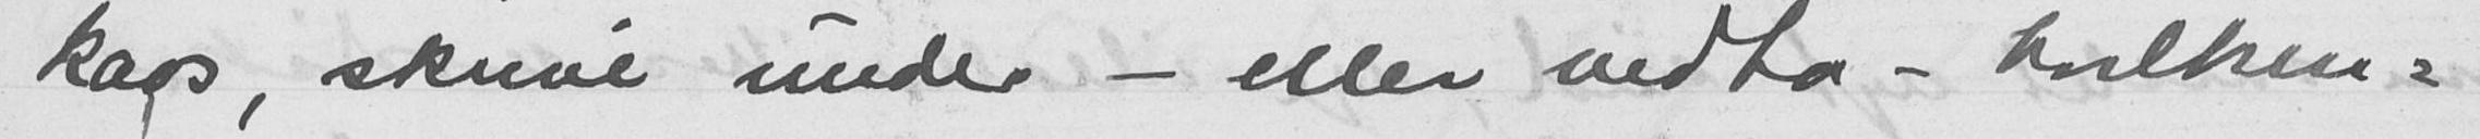kaos, skrive under - eller vedta - hvilken-
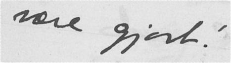være gjort."
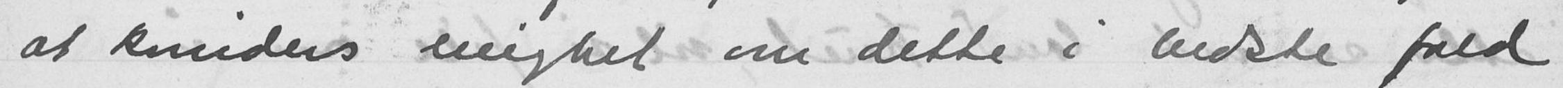at kvinders enighet om dette i bedste fald
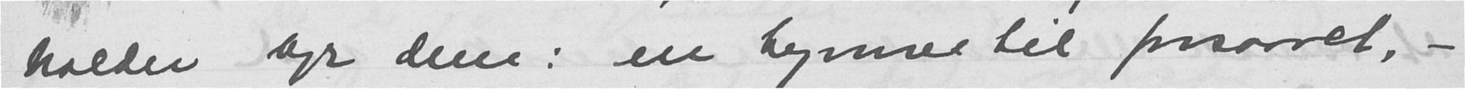holder byr dem; en kjenner til presset, -
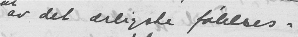av det ærligste følelses-
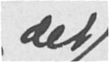det
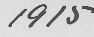1915
